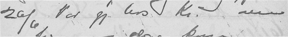26/6 Var jeg hos Kr. om
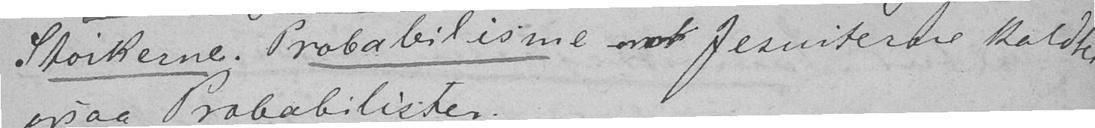Stoikerne. Probabilisme med Jesuiterene kaldtes
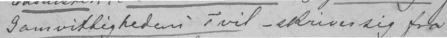Samvittighedens Tvil – skrive sig fra
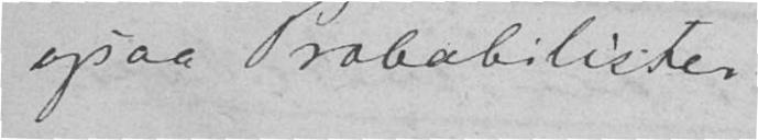ogsaa Probabilister.
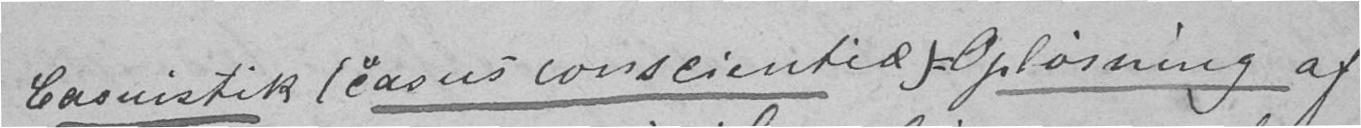Casuistik (casus conscientiæ) = Opløsning af
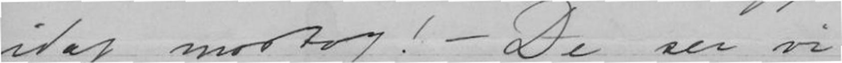idag modtog! - De ser vi
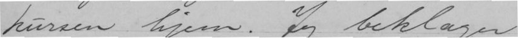kursen hjem. Jeg beklager
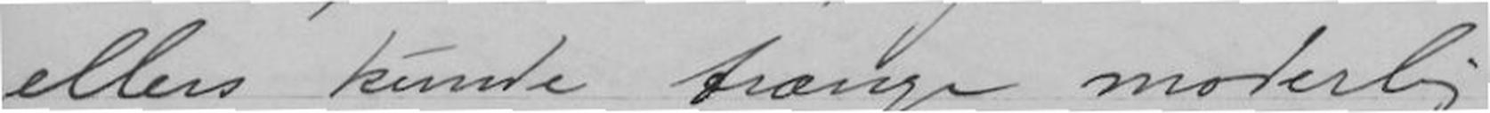ellers kunde trænge moderlig
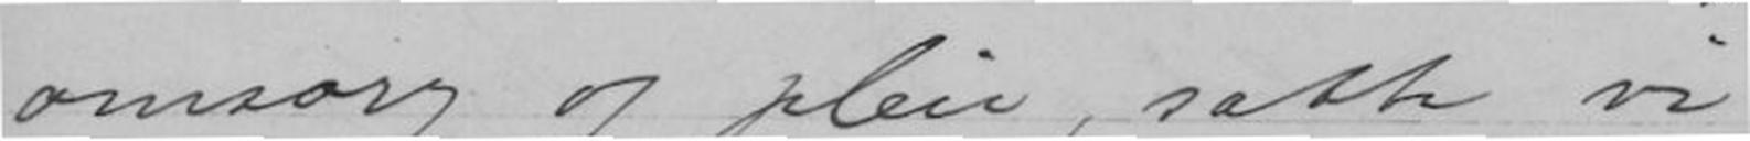omsorg og pleie, satte vi
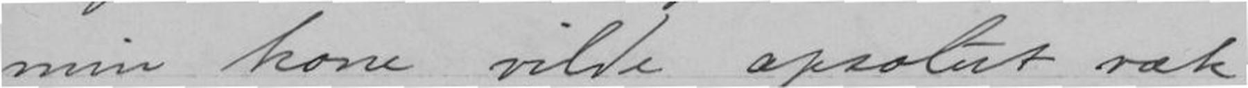min kone vilde apsolut væk
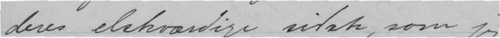deres elskværdige sidste, som jeg
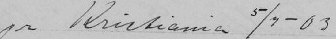pr Kristiania 5/7-03
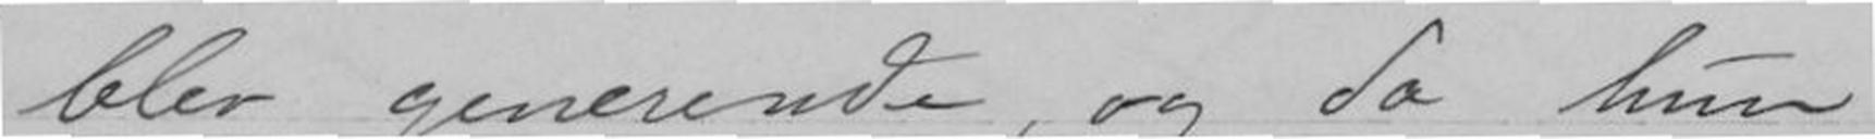blev generende, og da hun
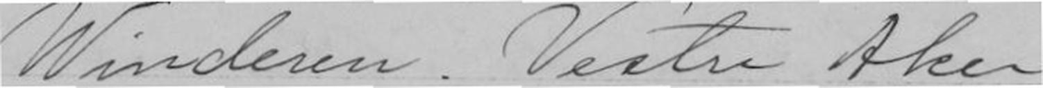Winderen, Vestre Aker
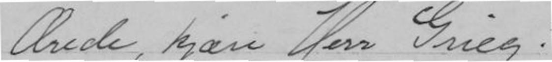Ærede, kjære Herr Grieg!
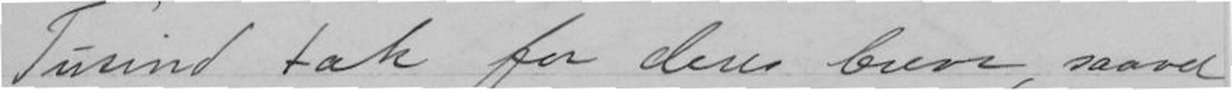Tusind tak for deres breve, saavel
